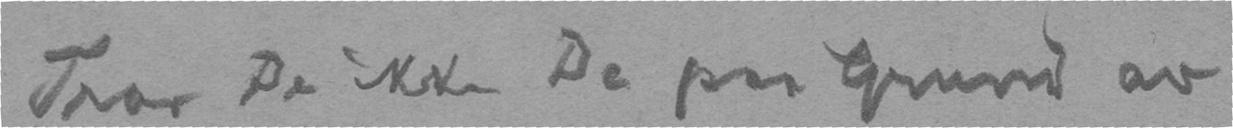Tror De ikke De paa Grund av
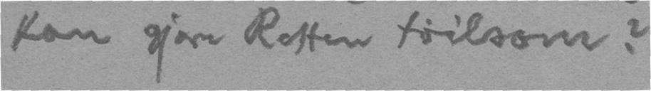kan gjøre Retten tvilsom?
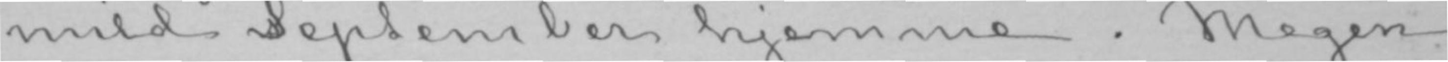mild sSeptember hjemme. Megen
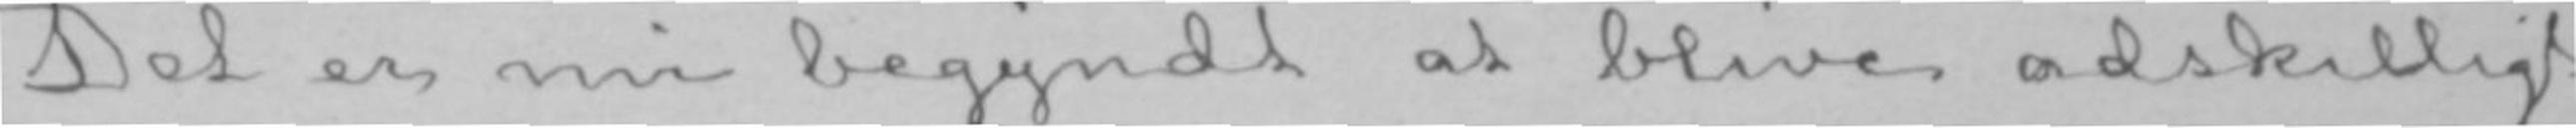Det er nu begyndt at blive adskilligt
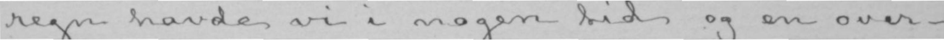regn havde vi i nogen tid og en over-
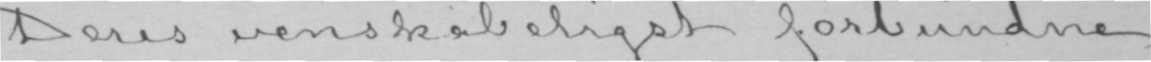Deres venskabeligst forbundne
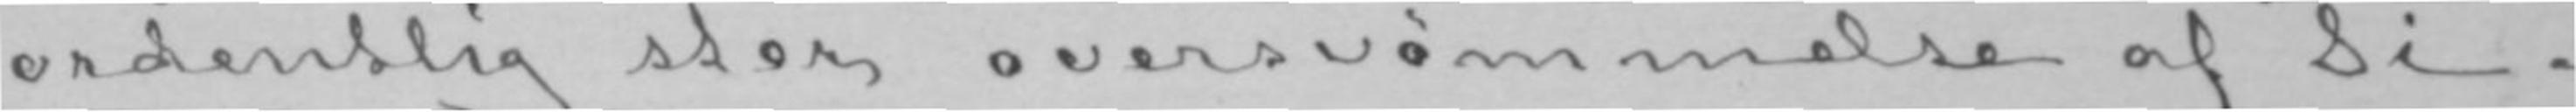ordentlig stor oversvømmelse af Ti-
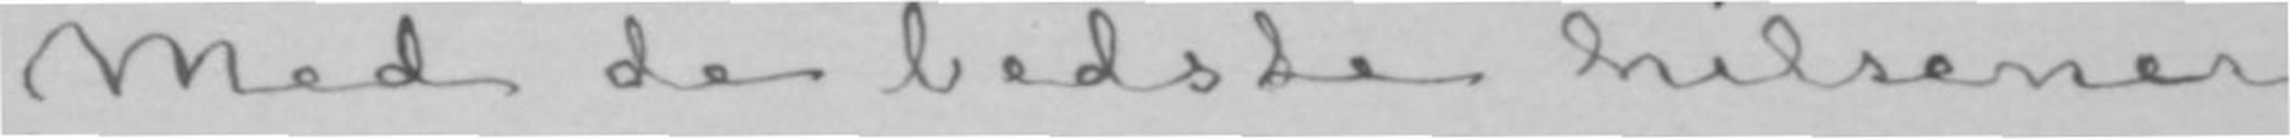Med de bedste hilsener
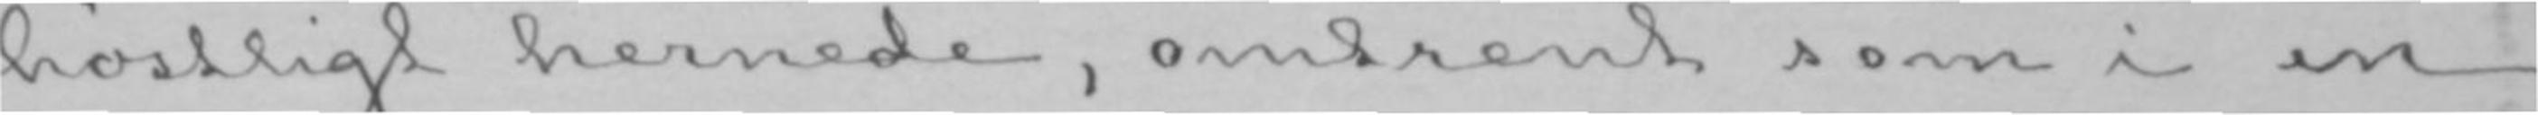høstligt hernede, omtrent som i en
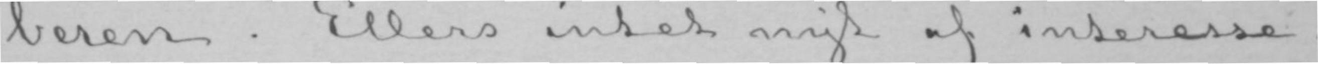beren. Ellers intet nyt af interesse.
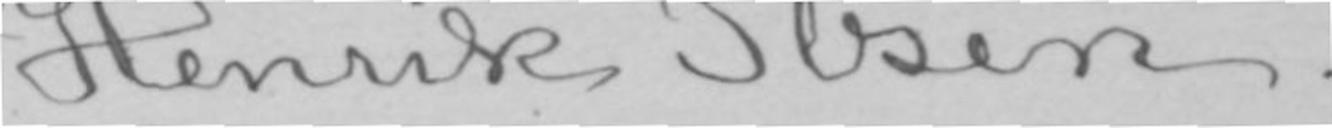Henrik Ibsen.
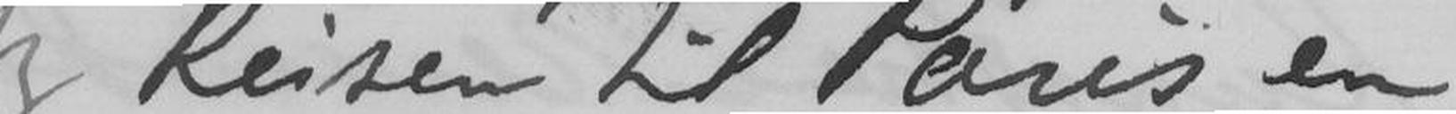Reisen til Paris en
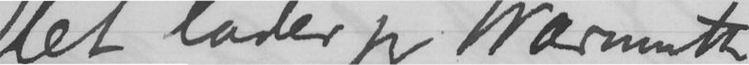let lader jeg Warmuth
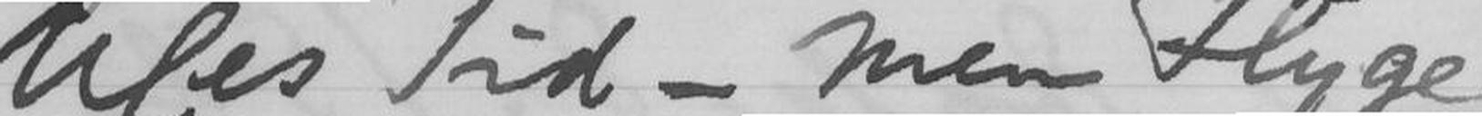uges Tid - men Flyge-
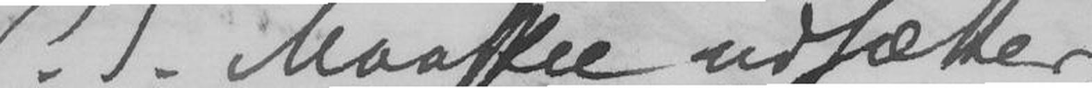P.S. Maaske udsætter jeg
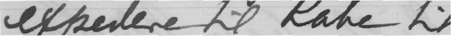expedere til Rabe til
